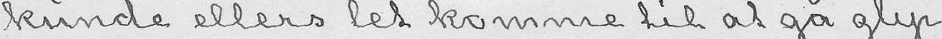kunde ellers let komme til at gå glip
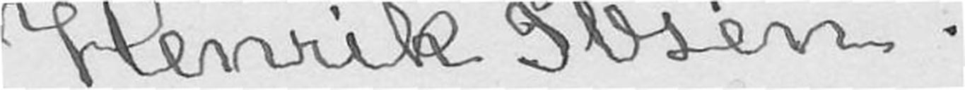Henrik Ibsen.
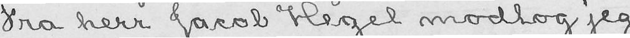Fra herr Jacob Hegel modtog jeg
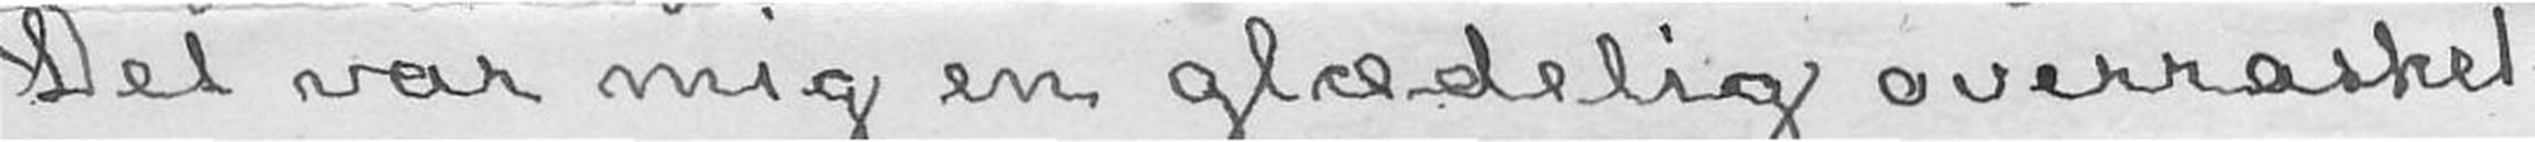Det var mig en glædelig overraskel-
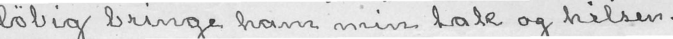løbig bringe ham min tak og hilsen.
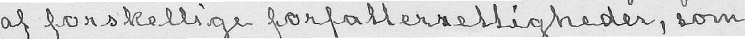af forskellige forfatterrettigheder, som
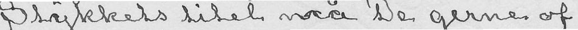Stykkets titel må De gerne of-
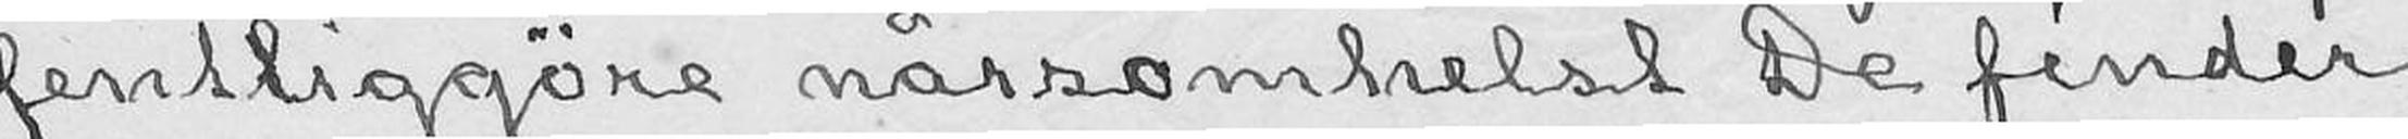fentliggøre nårsomhelst De finder
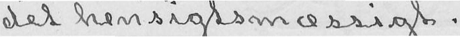det hensigtsmæssigt.
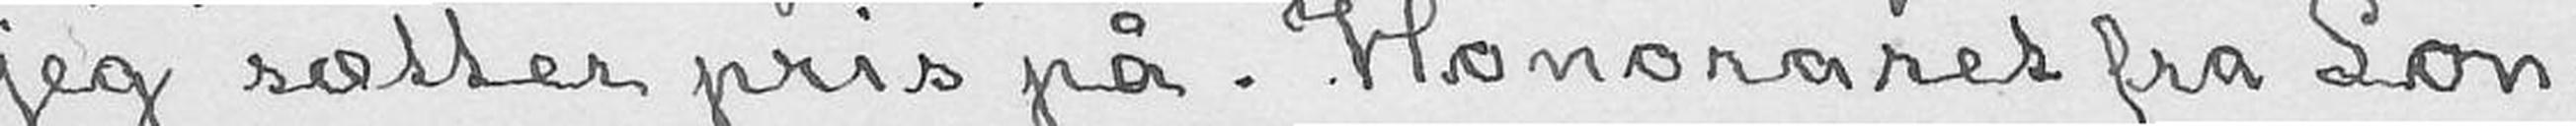jeg sætter pris på. Honoraret fra Lon-
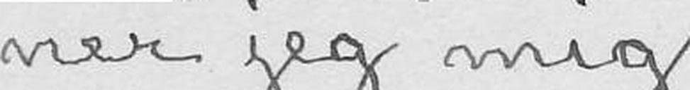ner jeg mig
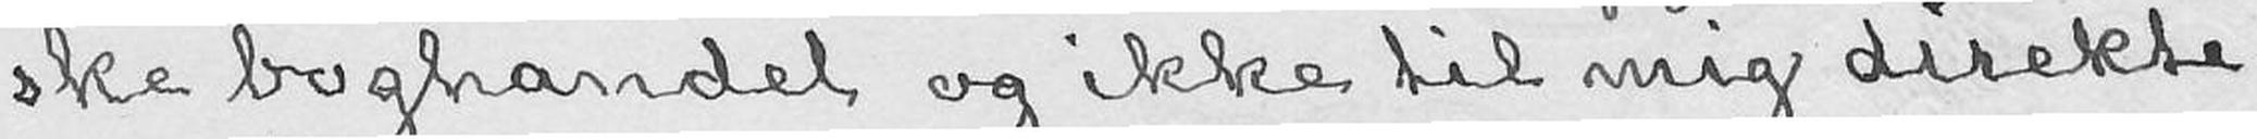ske boghandel og ikke til mig direkte.
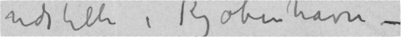udstille i Kjøbenhavn –
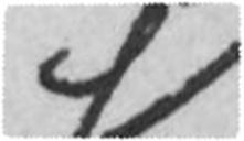4)
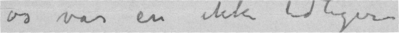os var en ikke tidligere
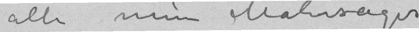alle mine Malersager
